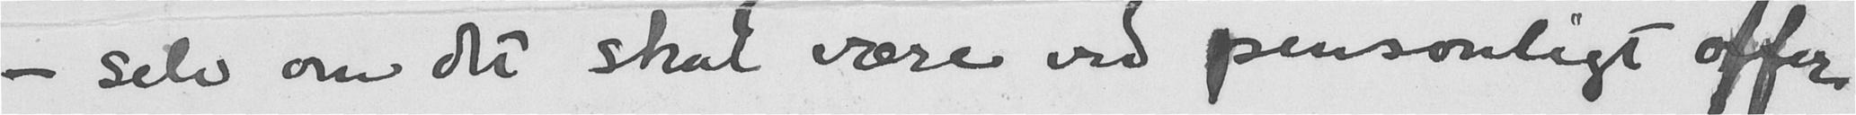- selv om det skal være ved personligt offer
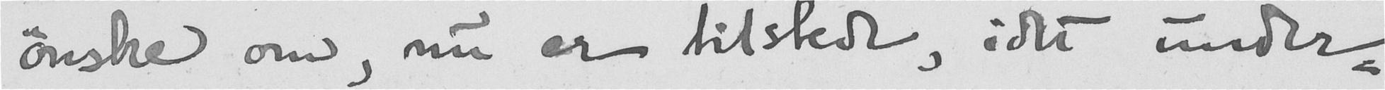ønske om, nu er tilstede, idet under-
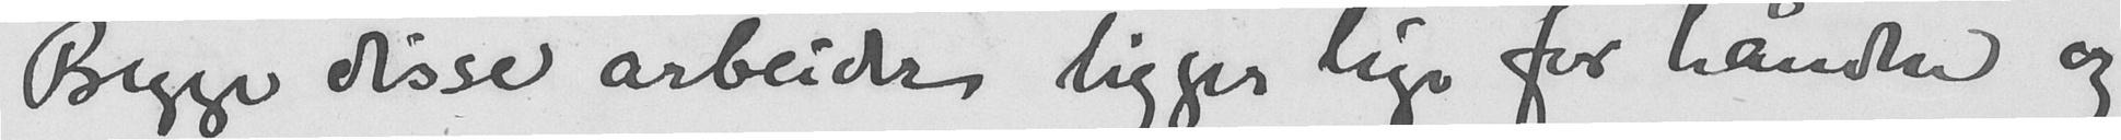Begge disse arbeider ligger lige for hånden og
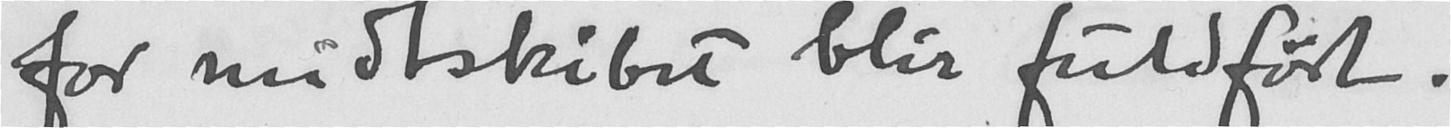for midtskibet blir fuldført.
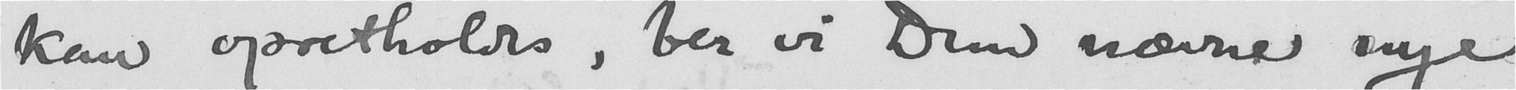kan opretholdes, ber vi Dem nævne nye
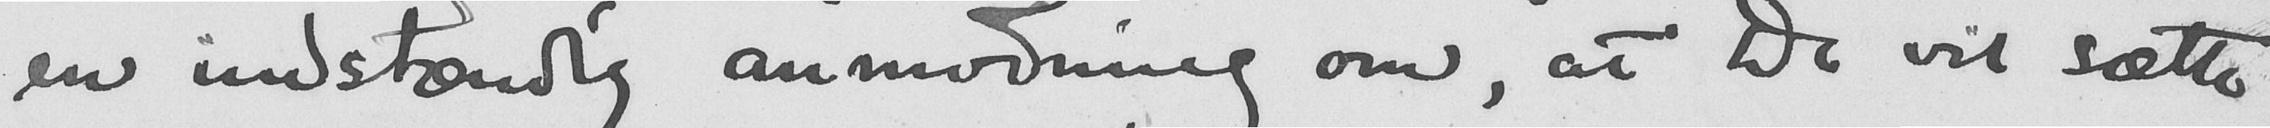en indstændig anmodning om, at De vil sætte
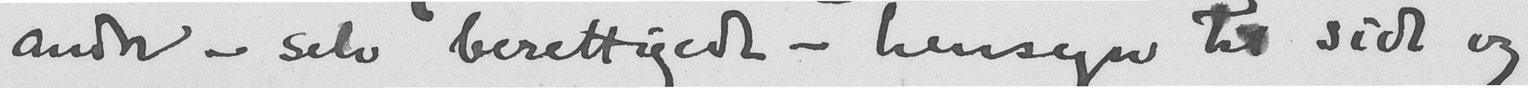andre - selv berettigede - hensyn til side og
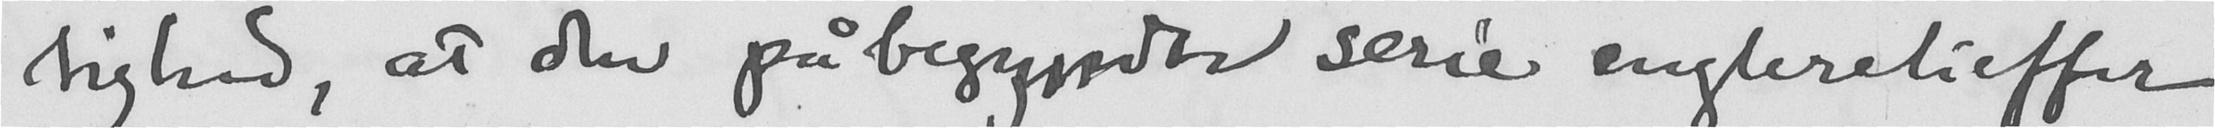tighed, at den påbegyndte serie englerelieffer
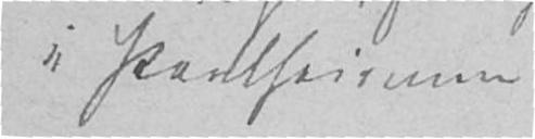i Pantheismen
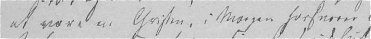at være en Christen, i Margen forsvarer
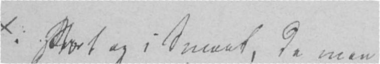i Stort og i Smaat, da man
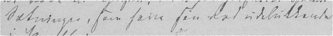sætninger, som have sin Ret udelukkende
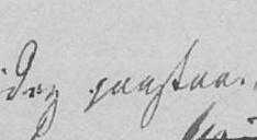dog paastaar,
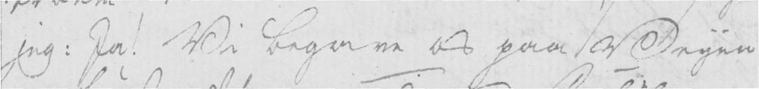jeg: Ja! Vi begave os paa Veyen
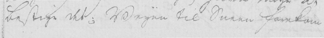bestige det; Veyen til Sneen forekom
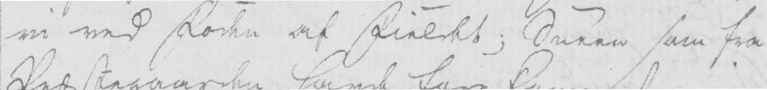vi ved Foden af Fieldet; Sneen som fra
